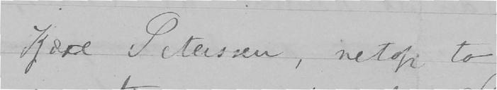Kjære Peterssen, netop to
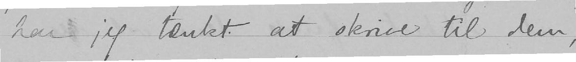har jeg tænkt at skrive til dem,
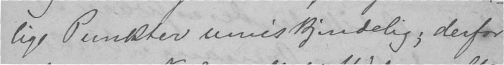lige Punkter umiskjendelig; derfor
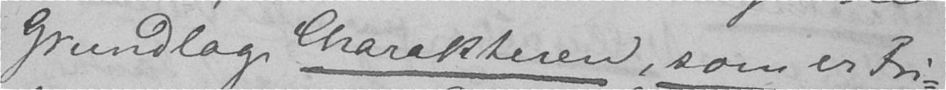Grundlag Charakteren, som er Fri-
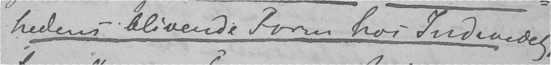hedens blivende Form hos Individet,
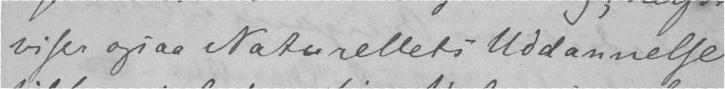viser ogsaa Naturellets Uddannelse
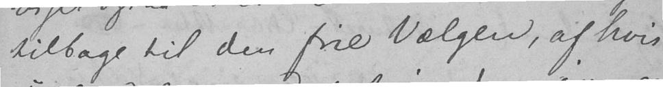tilbage til den frie Vælgen, af hvis
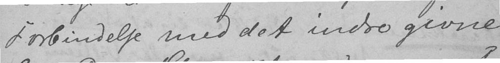Forbindelse med det indre givne
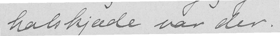halskjæde var der.
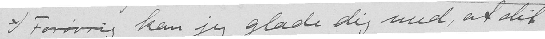x) Forøvrig kan jeg glæde dig med, at dit
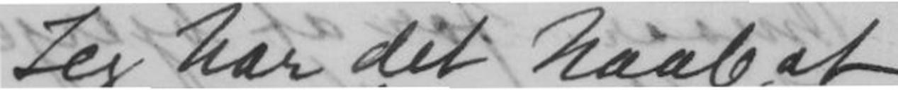Jeg har det haab, at
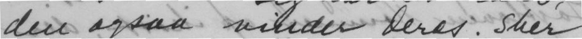den ogsaa vinder Deres. Sker
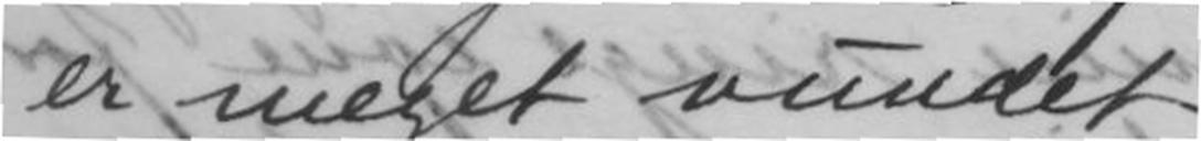er meget vundet.
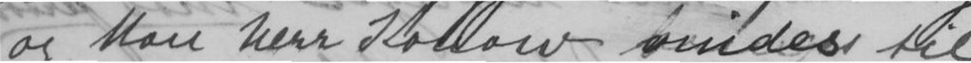og kan herr Konow vindes til
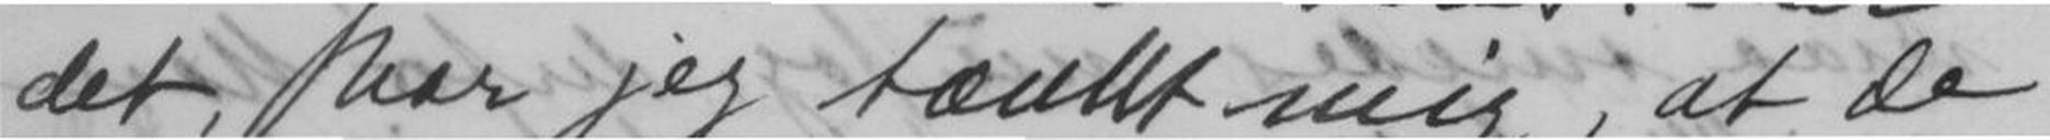det, har jeg tænkt mig, at De
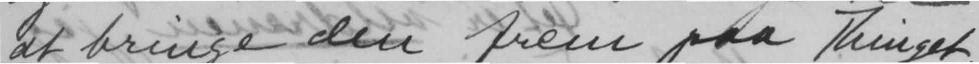at bringe den frem paa Thinget,
